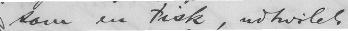som en Fisk, udhvilet,
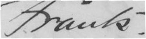Frants.
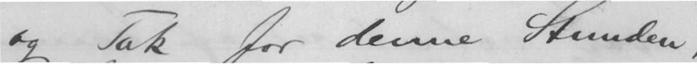og Tak for denne Stunden!
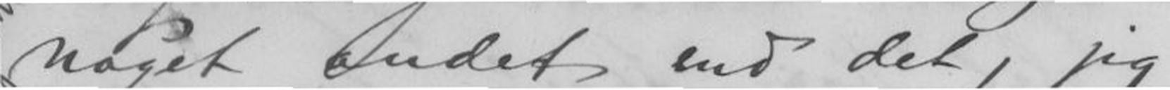noget andet end det, jeg
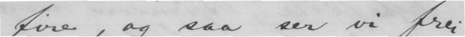fire, og saa ser vi frei-
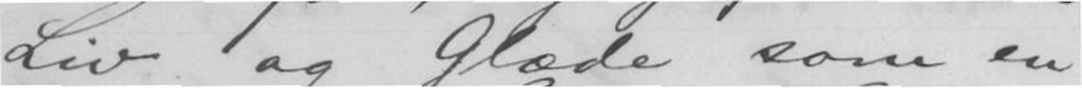Liv og Glæde som en
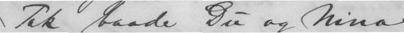Tak baade Du og Nina
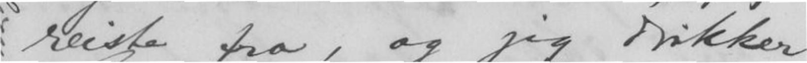reiste fra, og jeg drikker
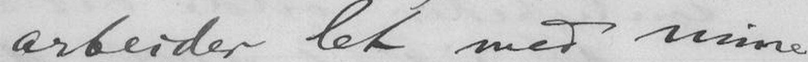arbeider let med mine
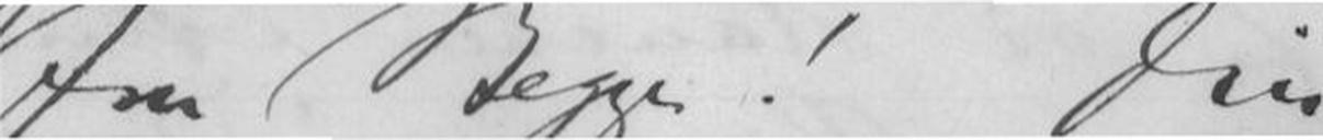fra Begge! Din
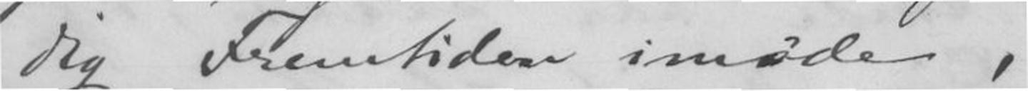dig Fremtiden imøde,
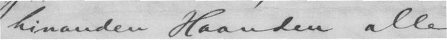hinanden Haanden alle
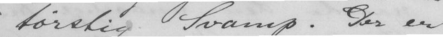tørstig Svamp. Der er
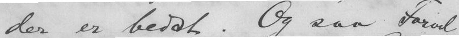hvad der er bedst. Og saa Farvel
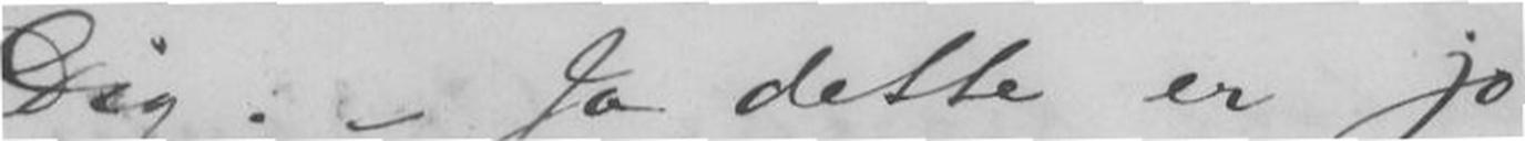Dig. - Ja dette er jo
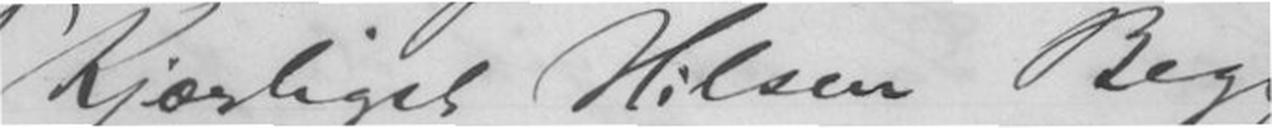Kjærligst Hilsen Begge
